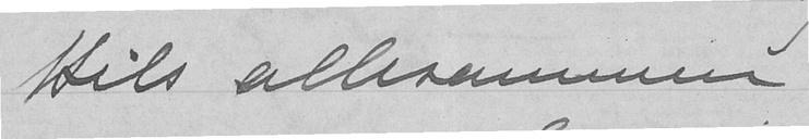Hils allesammen
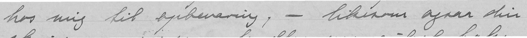hos mig til opbevaring, - likesom ogsaa din
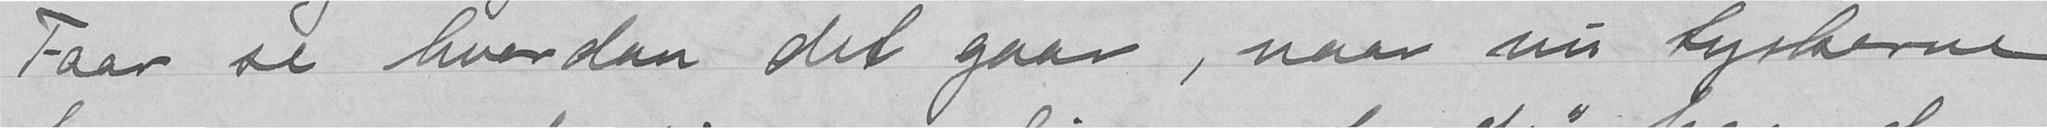Faar se hvordan det gaar, naar nu tyskerne
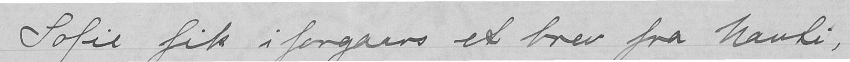Sofie fik i forgaars et brev fra Nanti,
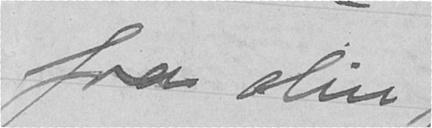fra din
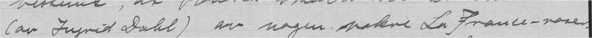(av Ingrid Dahl) av nogen vakre La France-roser.
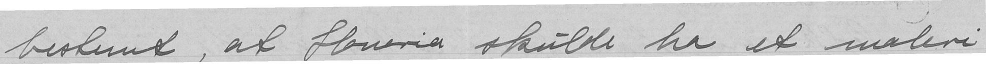bestemt, at Honoria skulde ha et maleri
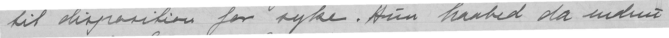til disposition for syke. Hun haabed da endnu
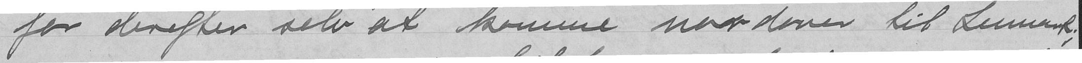for derefter selv at komme nordover til Lennart;
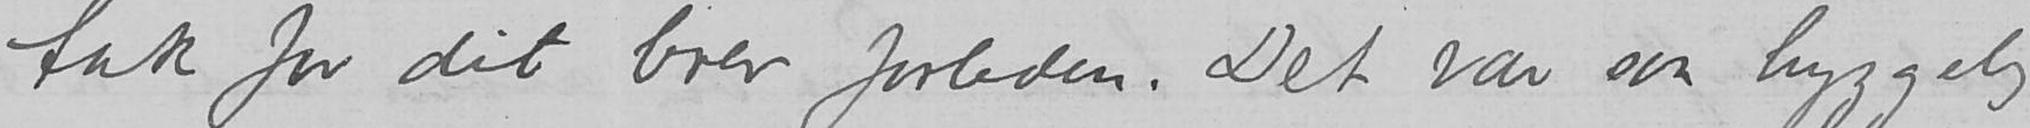tak for dit brev forleden. Det var saa hyggelig
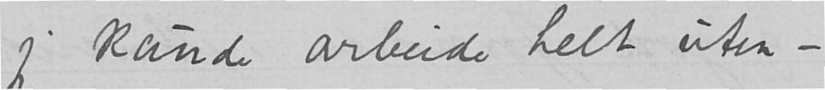jeg kunde arbeide helt uten –.
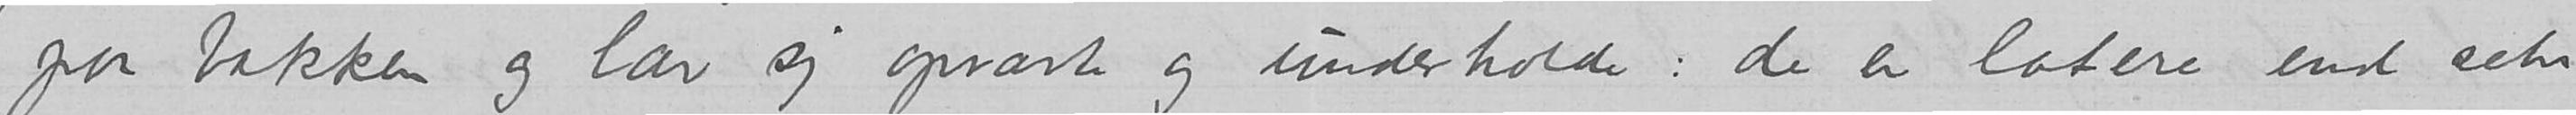paa bakken og lar sig opvarte og underholde: de er latere end
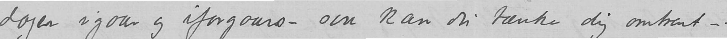dager igaar og iforgaars – saa kan du tænke dig omtrent
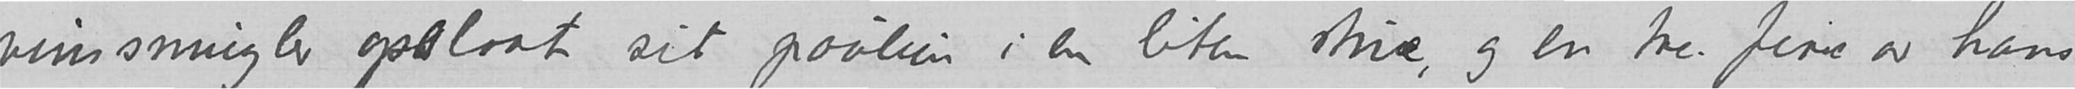vinssmugler opslaat sit poulien i en liten stue, og en tre, fire av hans
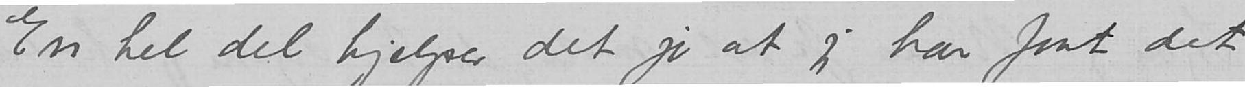En del del hjelper det jo at jeg har faat det
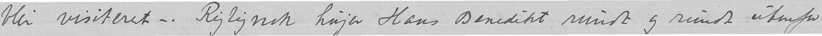blir visiteret –. Rigtignok hujer Hans Benedikt rundt og rundt utenfor
